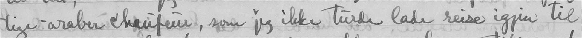tige araber chaufeur, som jeg ikke turde lade reise igjen til
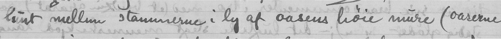lunt mellem stammerne i ly af aasens høie mure (oaserne
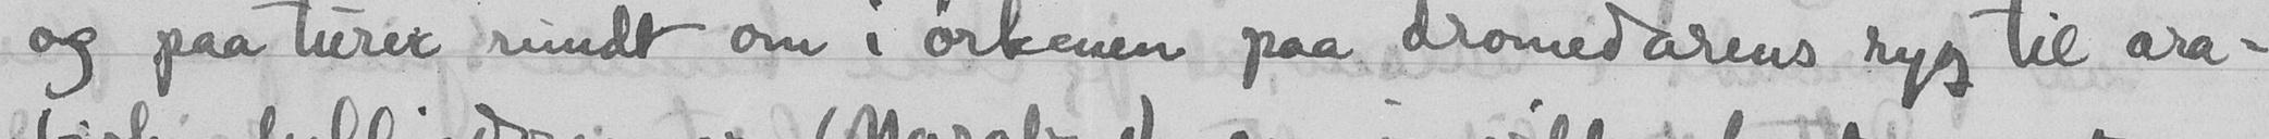og paa turer rundt om i ørkenen paa dromedarens ryg til ara-
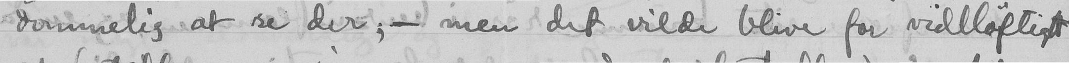dommelig at se der; - men det vilde blive for vidtløftidt
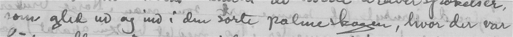som gled ud og ind i den sorte palmeskogen, hvor der var
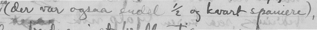(der var ogsaa endel 1/2 og kvart spaniere),
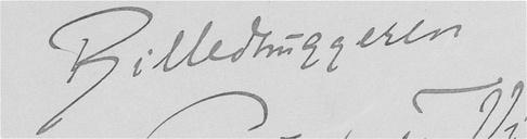Billedhuggeren
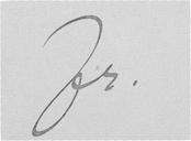fr.
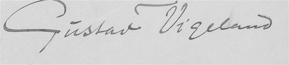Gustav Vigeland
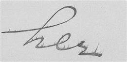her
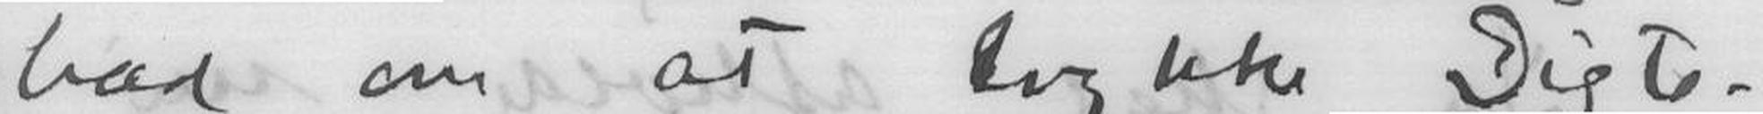bad om at trykke Digte-
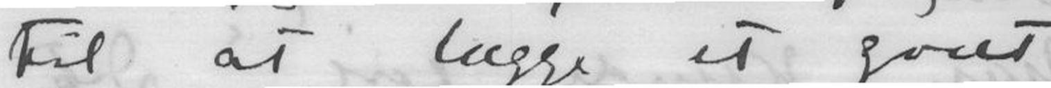til at lægge et godt
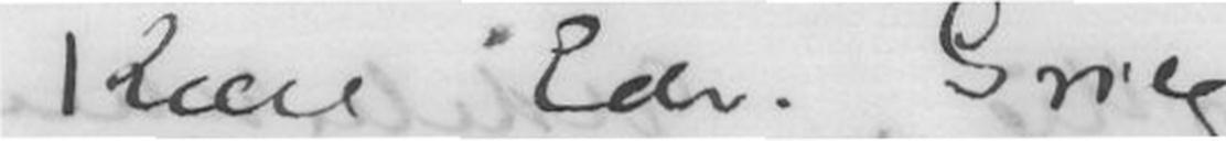Kære Edv. Grieg!
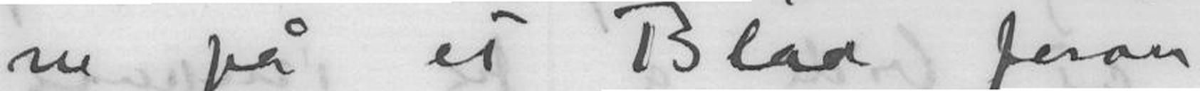ne på et Blad foran
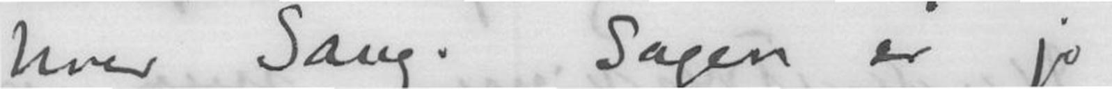hver Sang. Sagen er jo
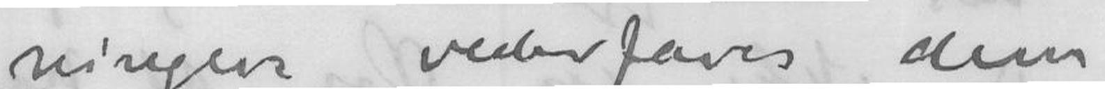ningen vederfares dem
